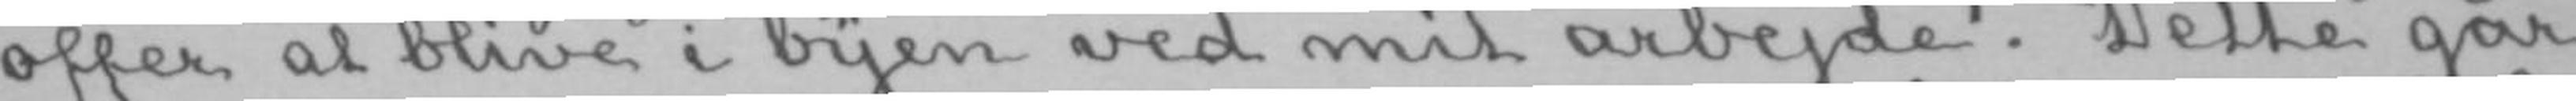offer at blive i byen ved mit arbejde. Dette går
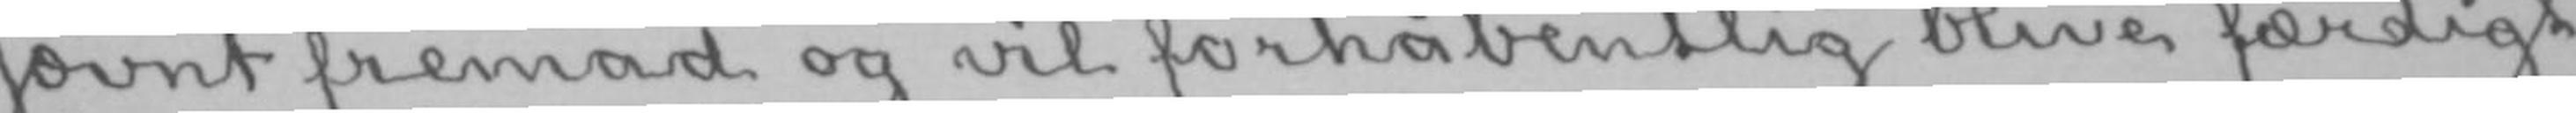jævnt fremad og vil forhåbentlig blive færdigt
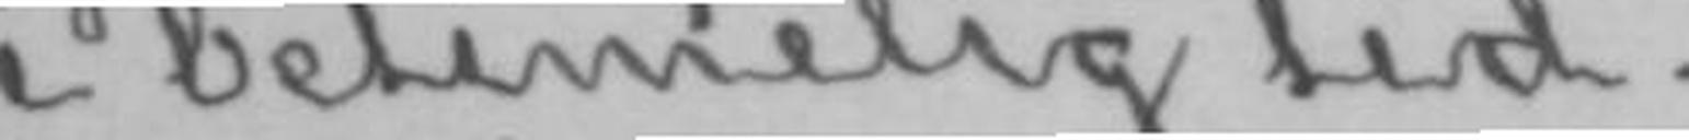i betimelig tid.
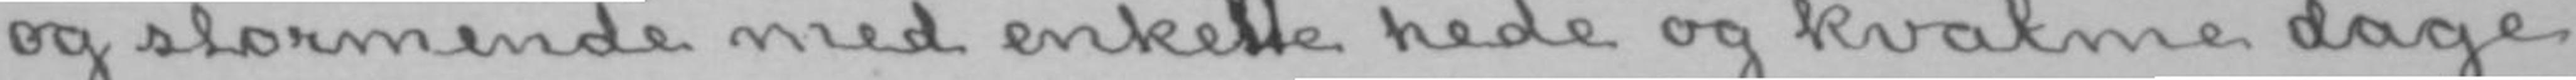og stormende med enketlte hede og kvalme dage
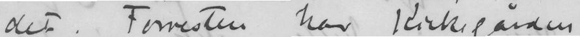det. Forresten har Kirkegården
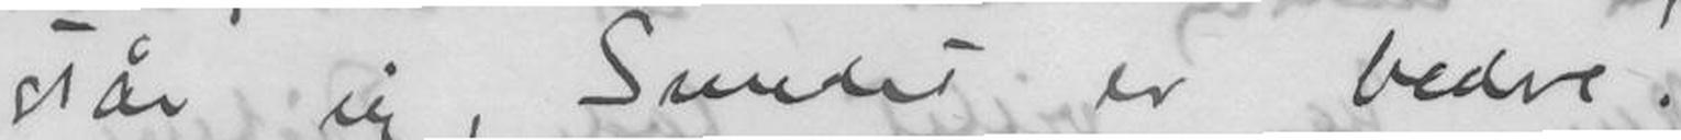står sig, Sundet er bedre.
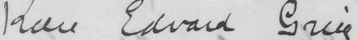Kære Edvard Grieg!
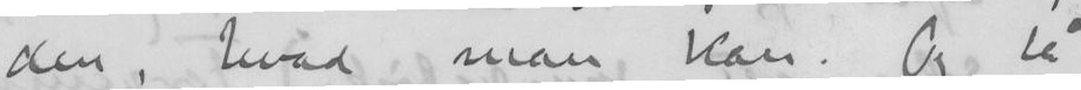den, hvad man kan. Og så
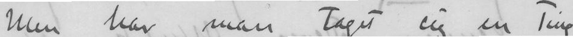Men har man Taget sig en Ting
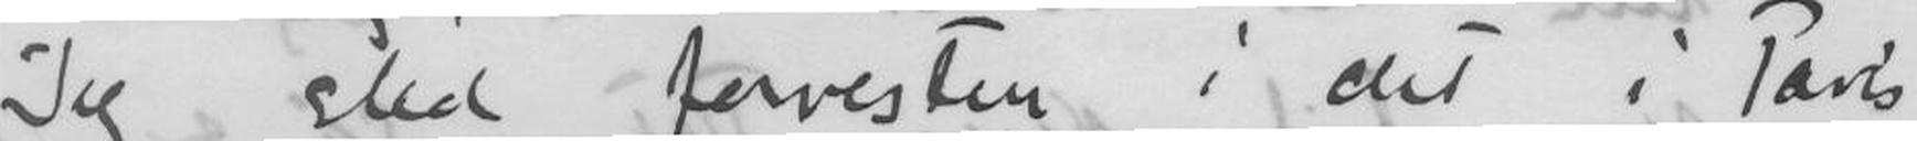Jeg sled forresten i det i Paris
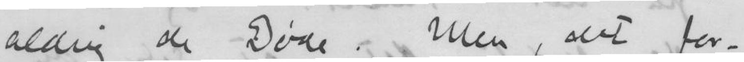aldrig de Døde. Men, det for-
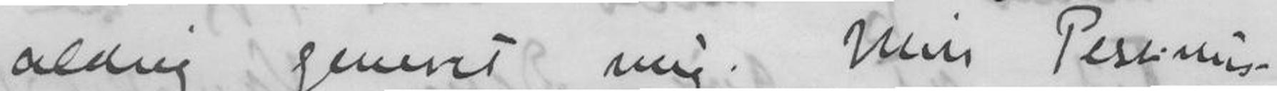aldrig generet mig. Min Pessimis-
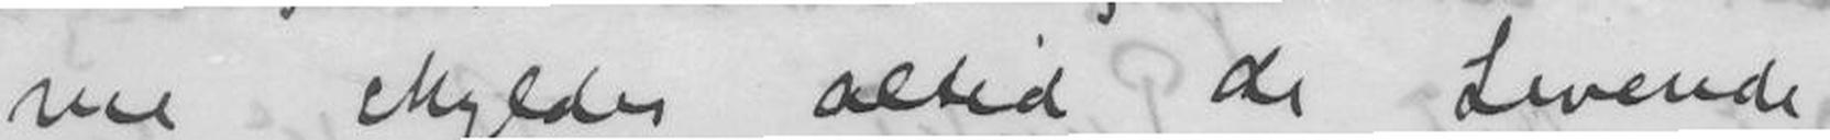me skylder altid de Levende,
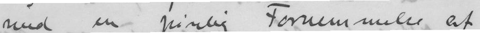med en pinlig Fornemmelse af,
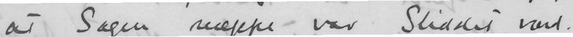at Sagen næppe var sliddet værd.
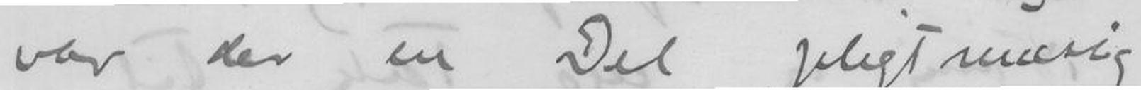var der en Del pligtmæsig
